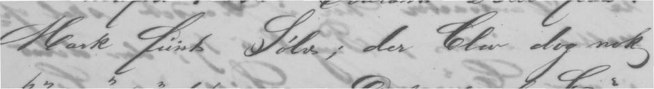Mark fint Sølv; der blev dog nok
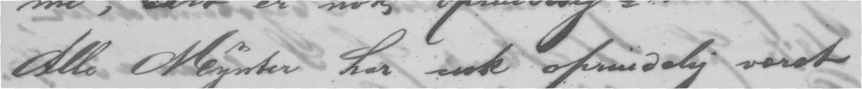Alle Mynter har nok oprindelig været
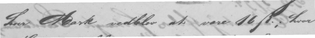hver Mark vedblev at være 16 ẞ; hver
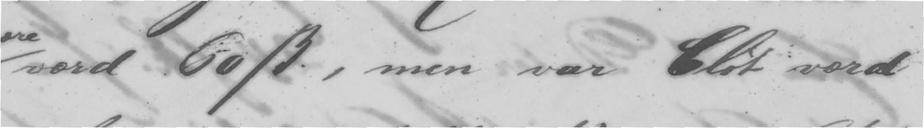værd 60 ẞ., men var blot værd
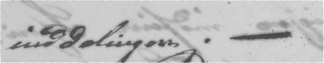inddelingen. -
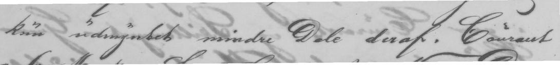kun udmyntet mindre Dale deraf. Courant
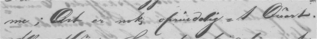me; Ort er nok oprindelig = 1 Quart.
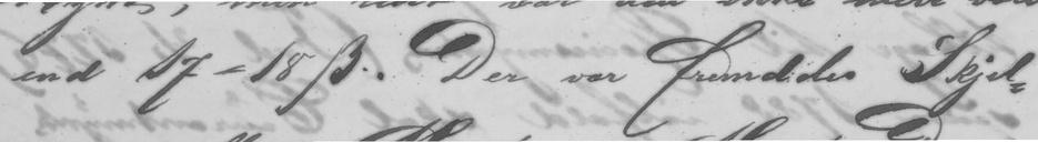end 17-18 ẞ. Den var fremdeles Skjil-
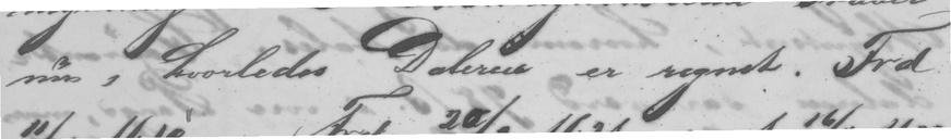nu, hvorledes Dalerne er regnet. Frd
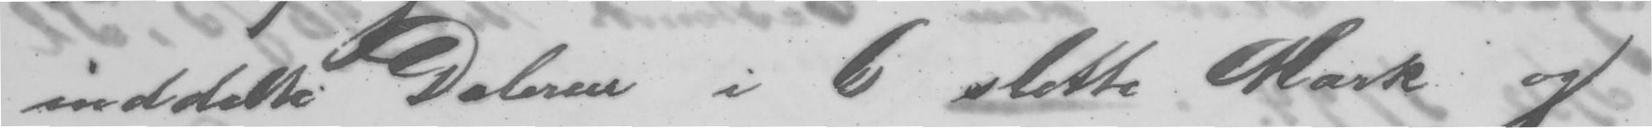inddelte Daleren i 6 slette Mark og
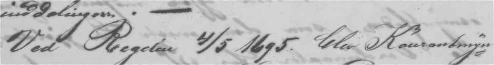Ved Regelen 4/5 1695. blev Kourantmyn-
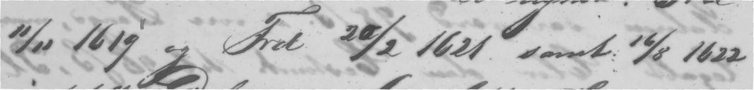11/11 1619 og Frd 20/2 1621 samt 16/8 1622
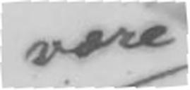være
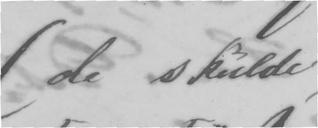(de skulde
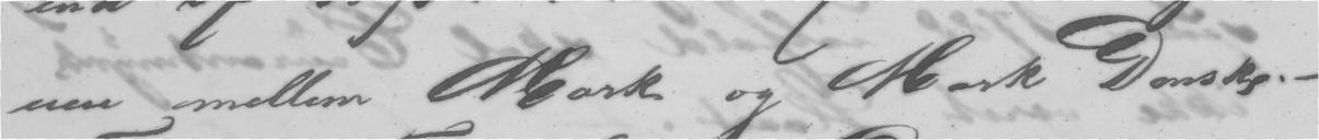nen mellem Mark og Mark Danske. -
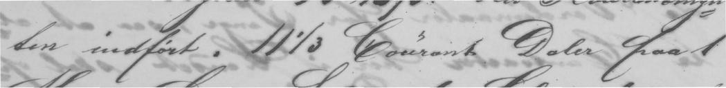ten indført. 11⅓ Courant Daler paa 1
